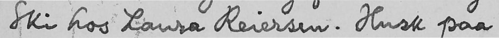Ski hos Laura Reiersen. Husk paa
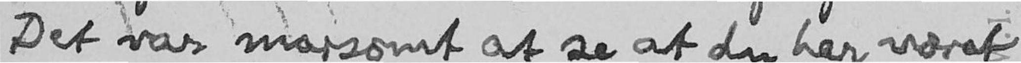Det var morsomt at se at du har været
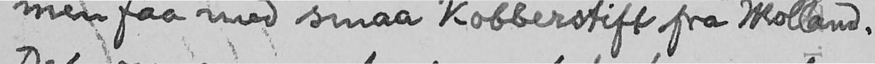men faa med smaa Kobberstift fra Molland.
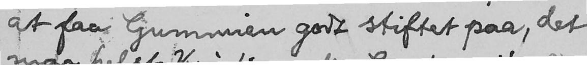at faa Gummien godt stiftet paa, det
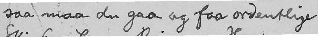saa maa du gaa og faa ordentlige
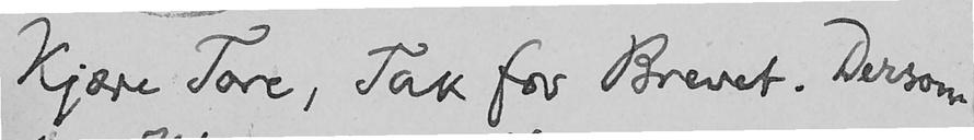Kjære Tore, Tak for Brevet. Dersom
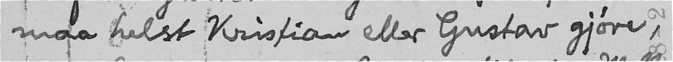maa helst Kristian eller Gustav gjøre,
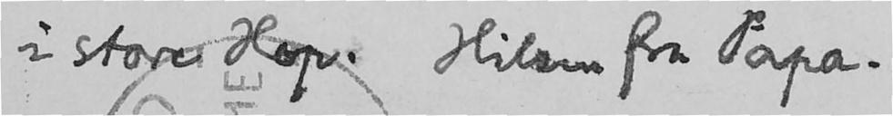i store Hop. Hilsen fra Papa.
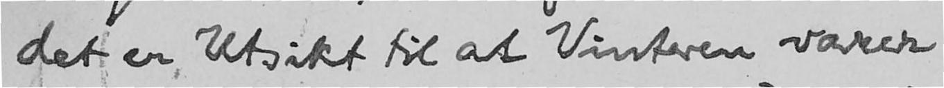det er Utsikt til at Vinteren varer
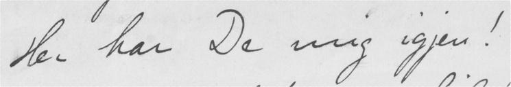Her har De mig igjen!
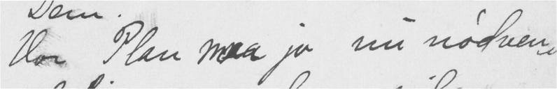Vor Plan maa jo nu nødven-
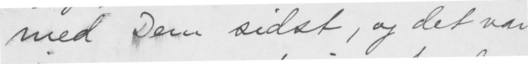med Dem sidst, og det var
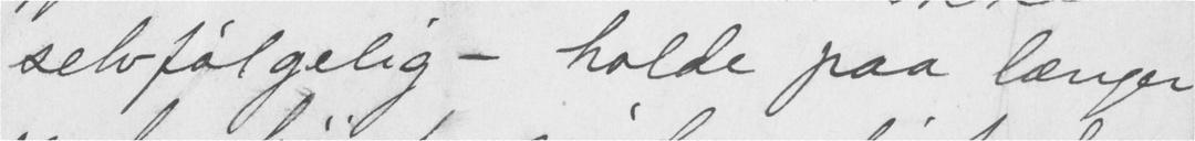selvfølgelig — holde paa længer
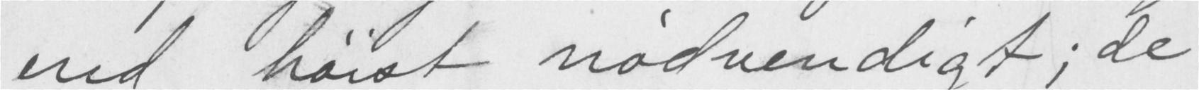end høist nødvendigt; de
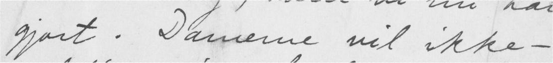gjort. Damerne vil ikke —
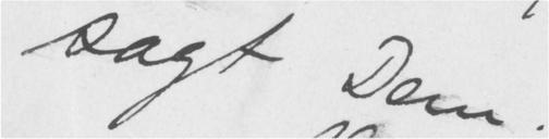sagt Dem.
